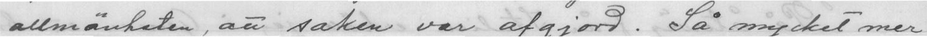allmänheten, att saken var afgjord. Så mycket mer
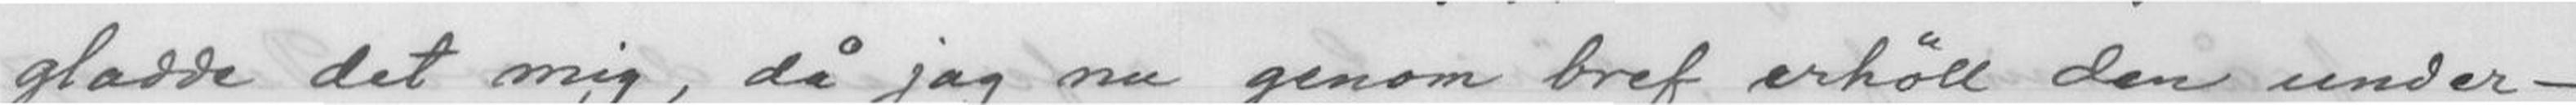gladde det mig, då jag nu genom bref erhöll den under-
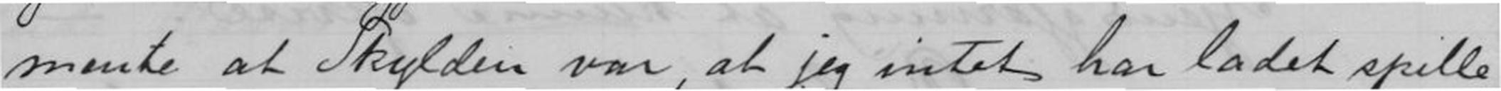nemte at Skylden var, at jeg intet har ladet spille
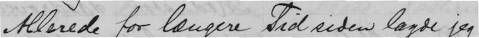Allerede for længere Tid siden lagde jeg
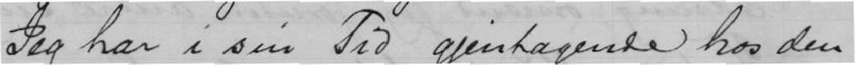Jeg har i sin Tid gjentagende hos den
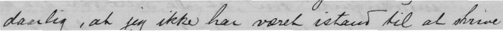daarlig, at jeg ikke har været istand til at skrive
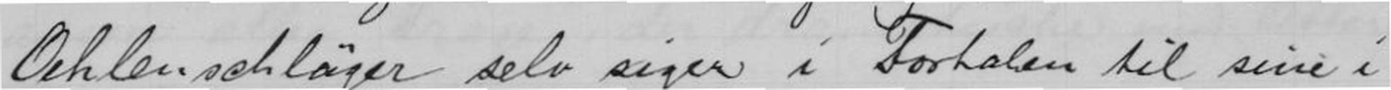Oehleenschläger selv siger i Fortalen til sine i
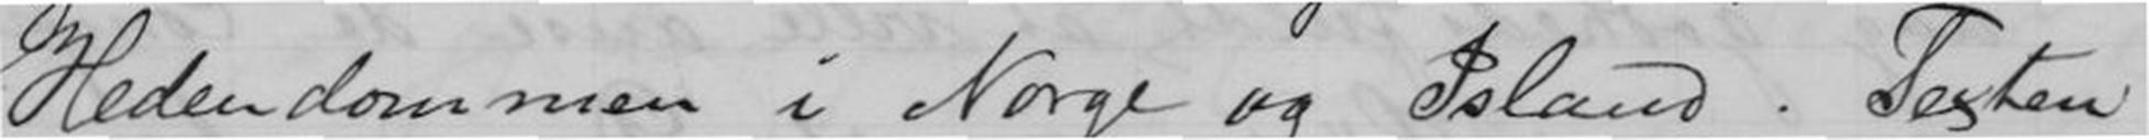Hedendommen i Norge og Island. Texten
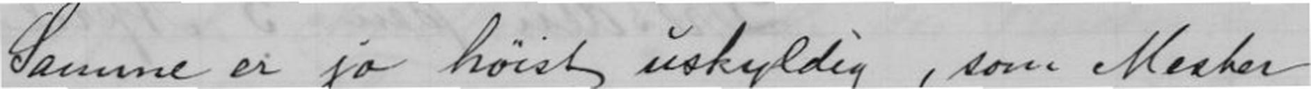Samme er jo høist uskyldig, som Mester
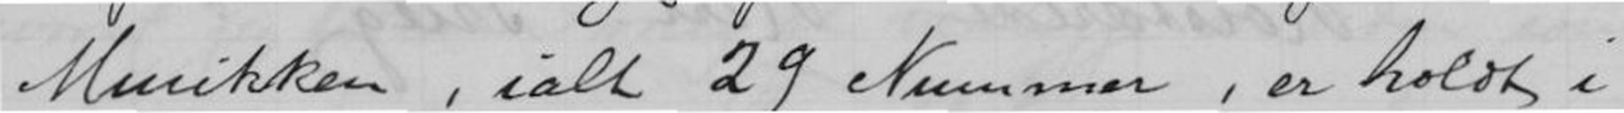Musikken, ialt 29 Nummer, er holdt i
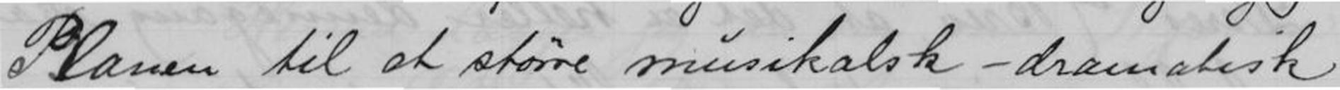Planen til et større musikalsk-dramatisk
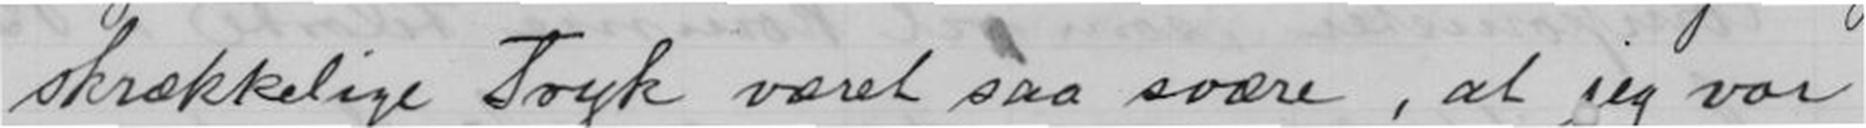skrekkelige Frygt været saa svære, at jeg var
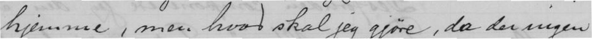hjemme, men hvad skal jeg gjøre, da der ingen
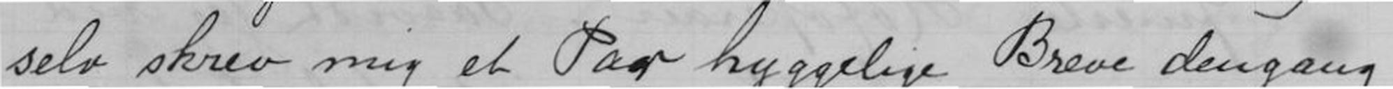selv skrev mig et Par hyggelige Breve dengang
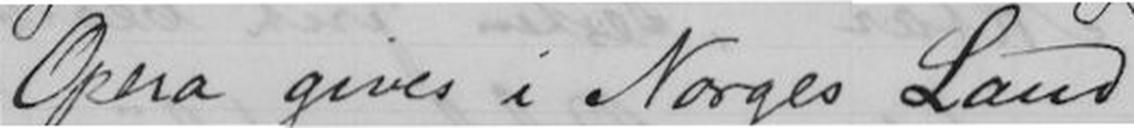Opera gives i Norges Land?
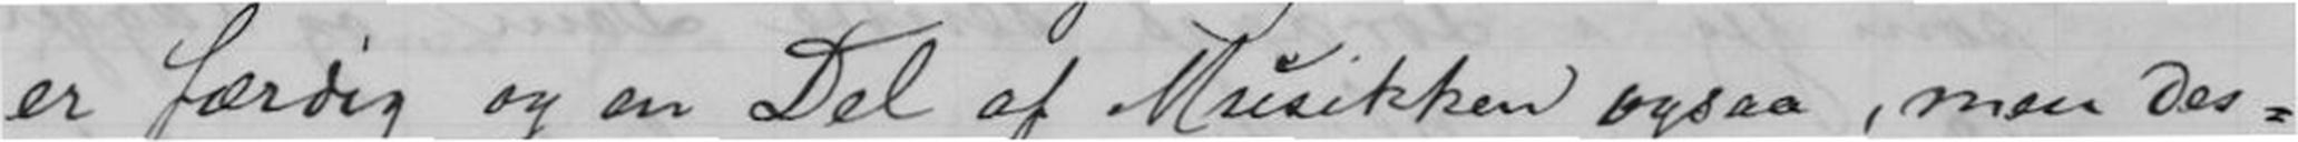er færdig og en Del af Musikken ogsaa, men des-
